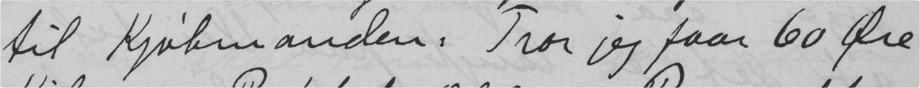til Kjøbmanden. Tror jeg faar 60 Øre
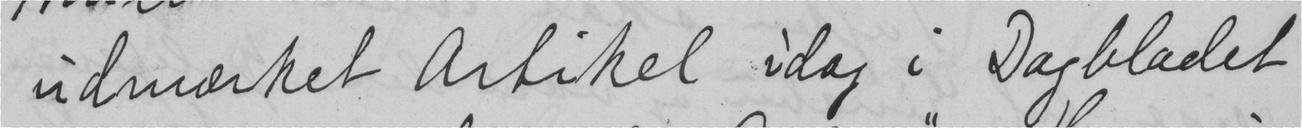udmærket Artikel idag i Dagbladet
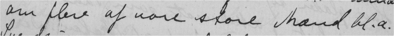om flere af vore store Mænd bl.a.
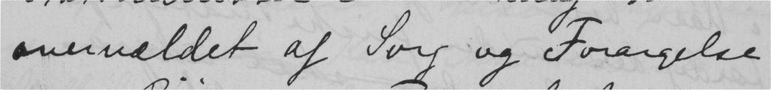overvældet af Sorg og Forargelse
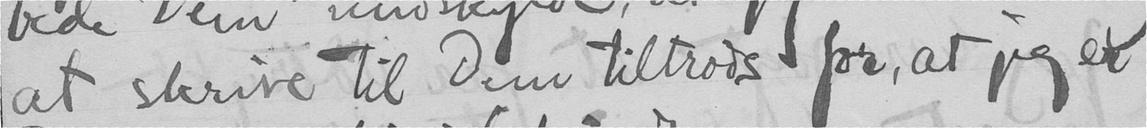at skrive til Dem tiltrods for, at jeg er
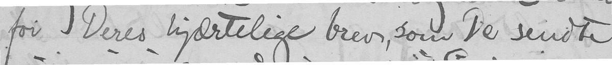for Deres kjærtelige brev, som De sendte
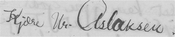Kjære Hr. Aslaksen.
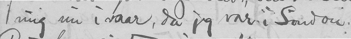mig nu i vaar, da jeg varre Sondon.
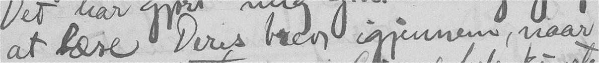at læse Deres brev igjennem, naar
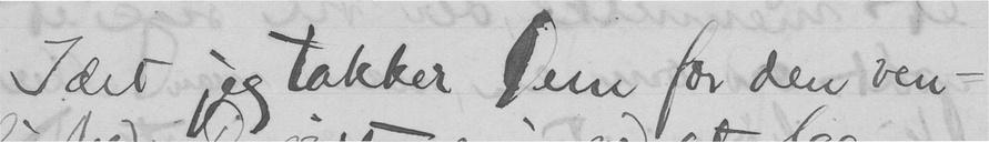I det jeg takker Dem for den ven-
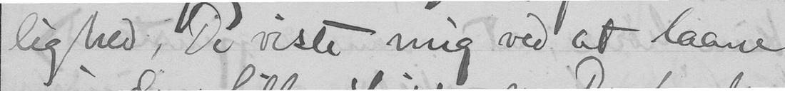lighed, De viste mig ved at laane
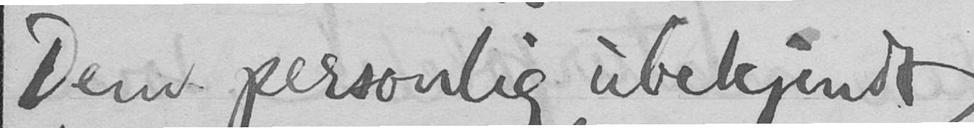Dem personlig ubekjendt
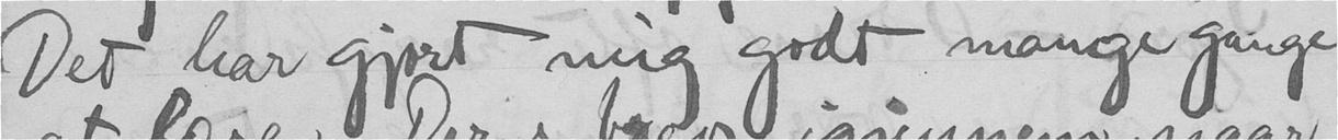Det har gjort mig godt mange gange
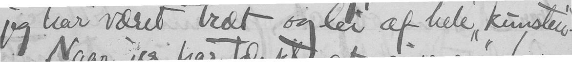jeg har været træt og lei af hele "kunsten".
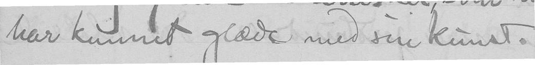har kunnet glæde - med sin kunst.
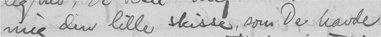mig den lille skisse, som De havde
